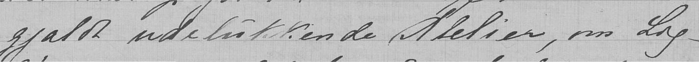gjaldt udelukkende Atelier, om Lig-
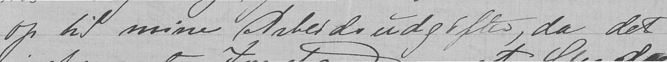op til mine Arbeidsudgifter, da det
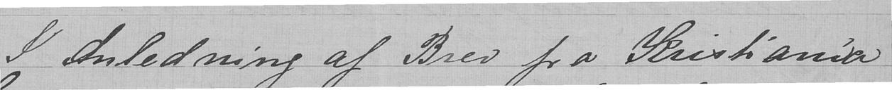I Anledning af Brev fra Kristiania
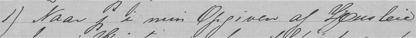1) Naar jeg i min Opgiven af Husleie
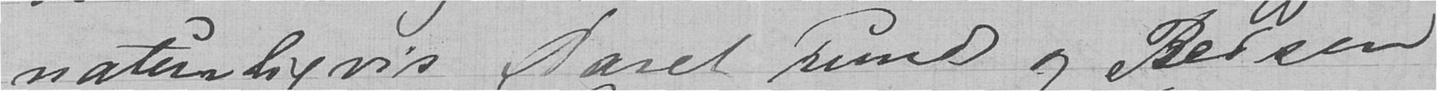naturligvis Aaret rundt og Reisen
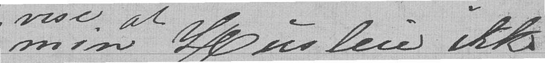min Husleie ikke
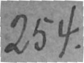254.
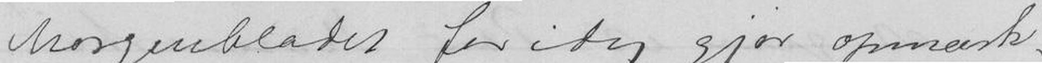Morgenbladet for idag gjør opmærk-
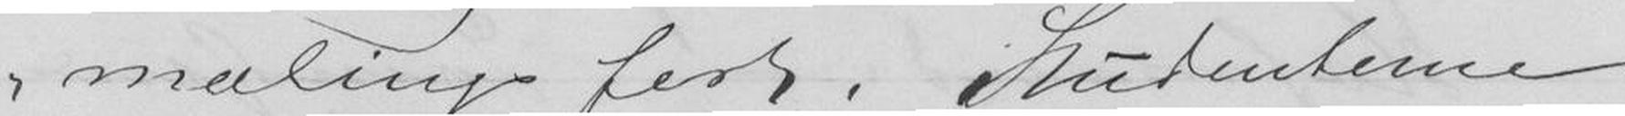maling fest. Studenterne
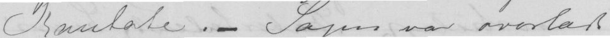Kantate. - Sagen var overladt
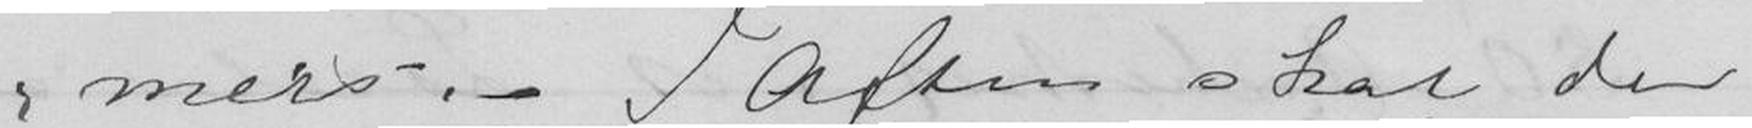mers. - I Aften skal der
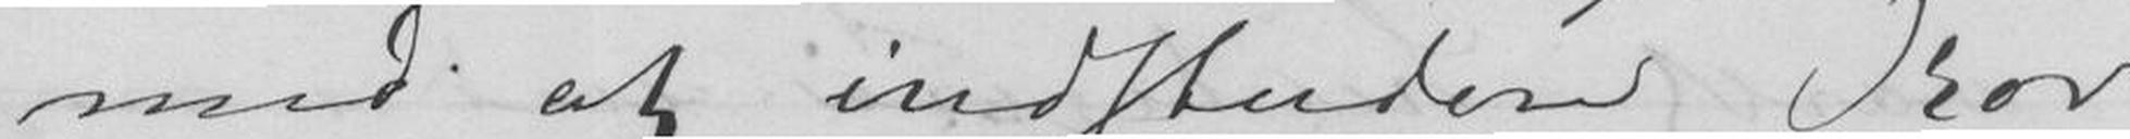med at indstudere Kor
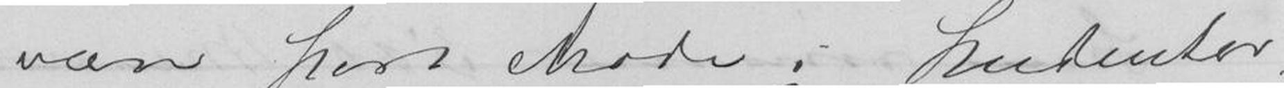være stort Møde i Studenter-
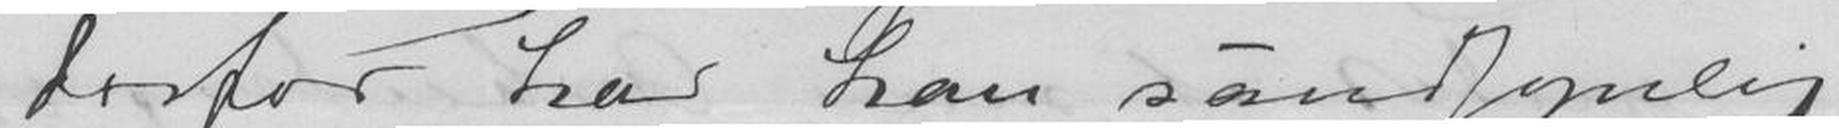derfor har han sandsynlig-
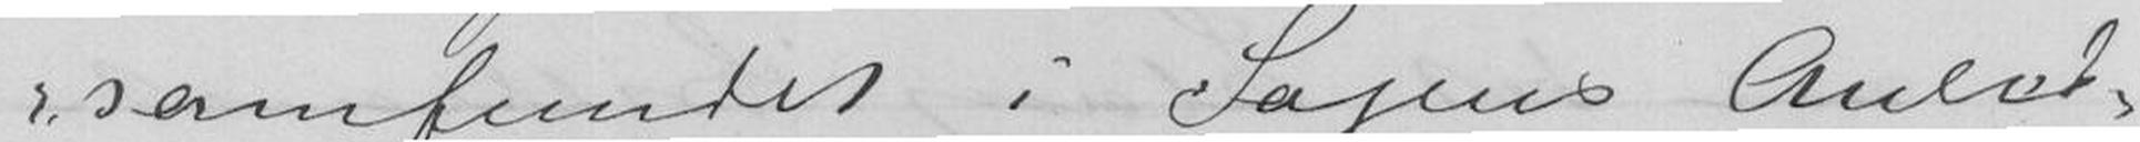samfundet i Sagens Anled-
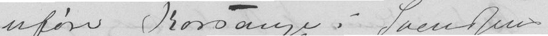uføre Korsange i Svendsens
